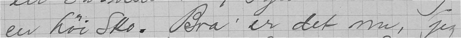en høi sko. Bra er det nu, jeg
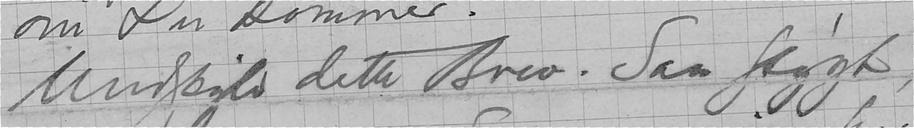Undskyld dette Brev. Saa stygt,
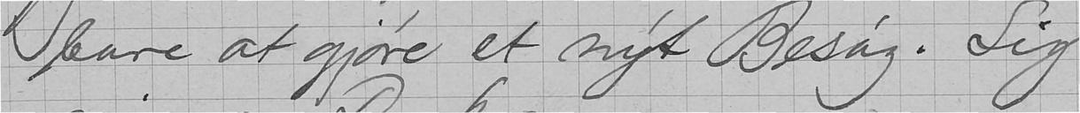bare at gjøre et nyt Besøg. Sig
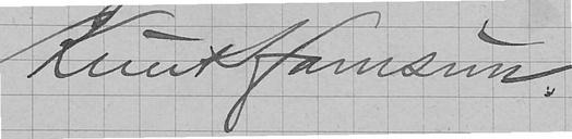Knut Hamsun.
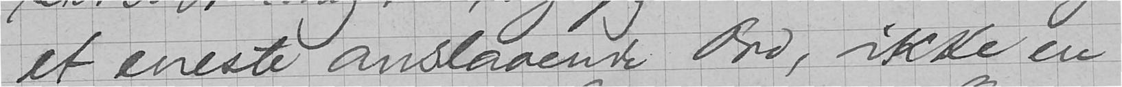et eneste anslaaende Ord, ikke en
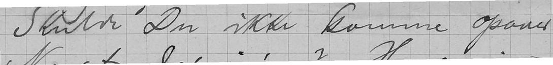Skulde Du ikke komme opover
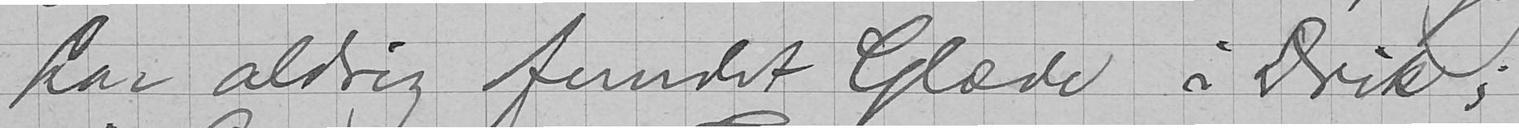har aldrig fundet Glæde i Drik;
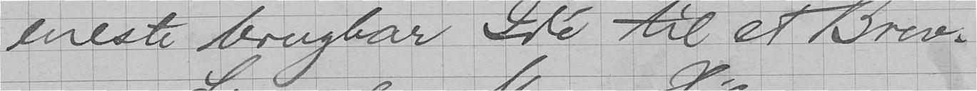eneste brugbar Ide til et Brev.
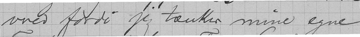vred fordi jeg tænker mine egne
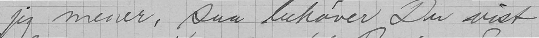jeg mener, saa behøver Du vist
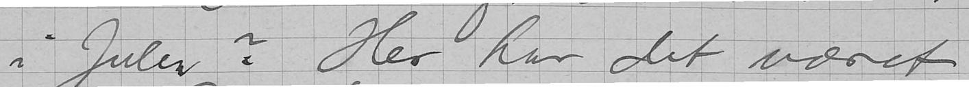i Julen? Her har det været
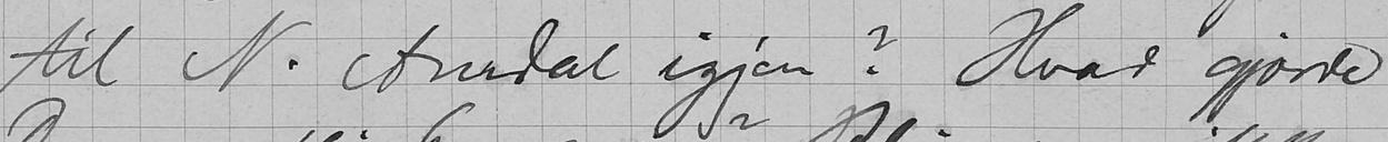til N. Aurdal igjen? Hvad gjorde
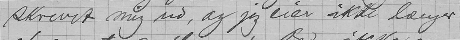skrevet mig ud, og jeg eier ikke længer
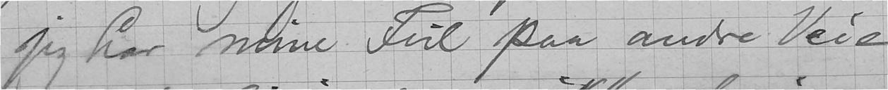jeg har mine Feil paa andre Veie
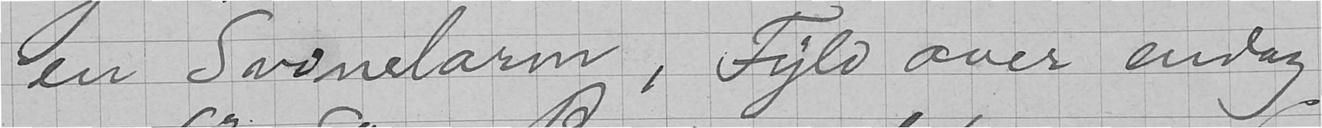en Svinelarm, Fyld over endog
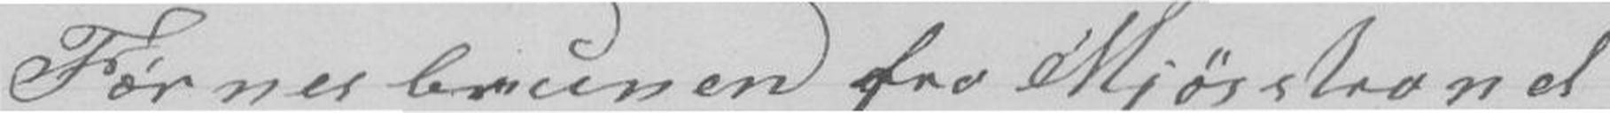Førnesbrunen fra Mjøsstrand
